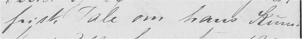frisk Tale om hans Kunst.
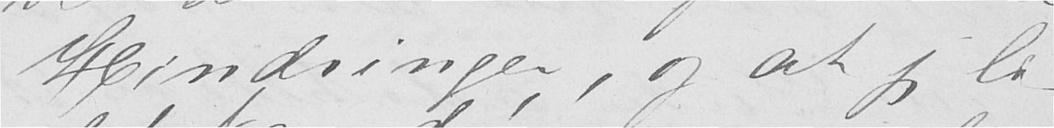Hindringer, og at jeg li-
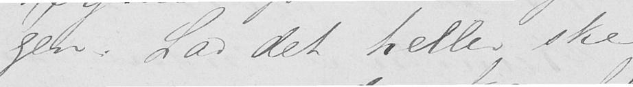gen. Lad det heller ske
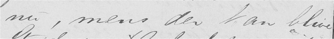nu, mens der kan blive
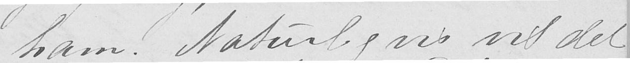ham! Naturligvis vil det
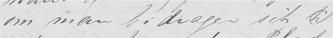om man bidrager sit til
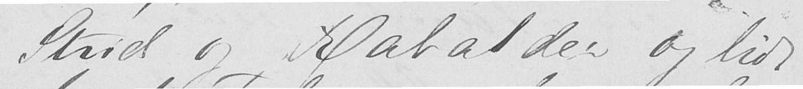Strid og Rabalder og lidt
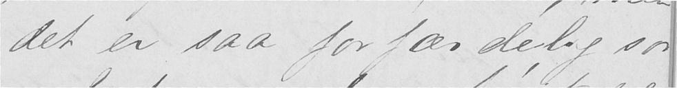det er saa forfærdelig sør-
